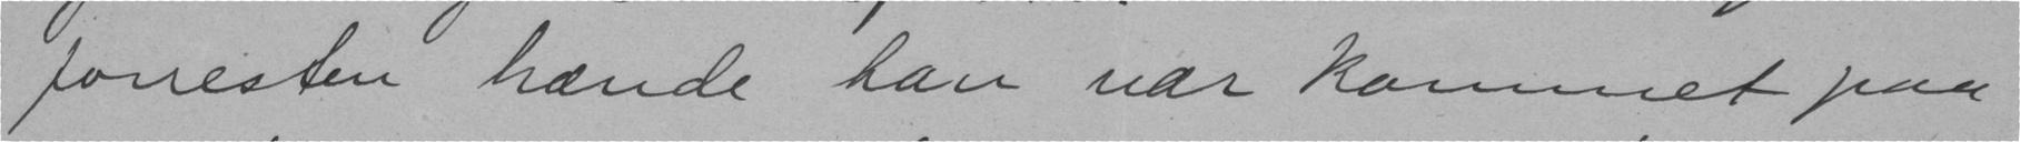foresten hænde han var kommet paa
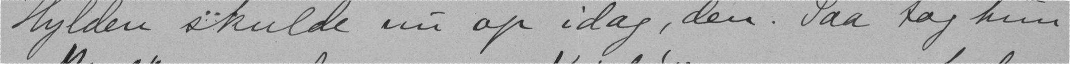Hylden skulde nu op idag, den. Saa tog hun
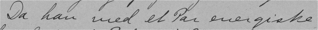Da han ved et Par energiske
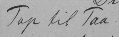Top til Taa.
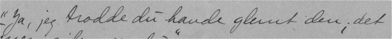"Ja, jeg trodde du havde glemt den; det
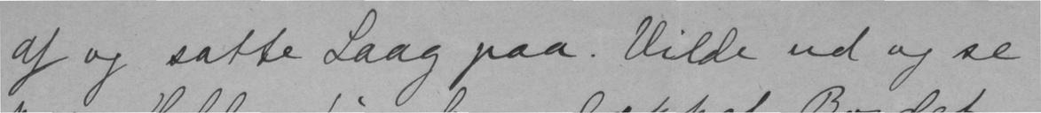af og satte Laag paa. Vilde ud og se
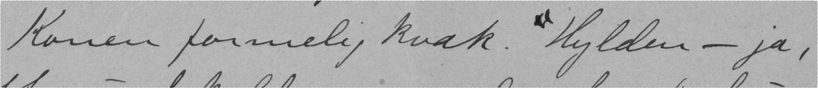Konen formelig kvak. "Hylden - ja,
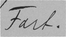Fart.
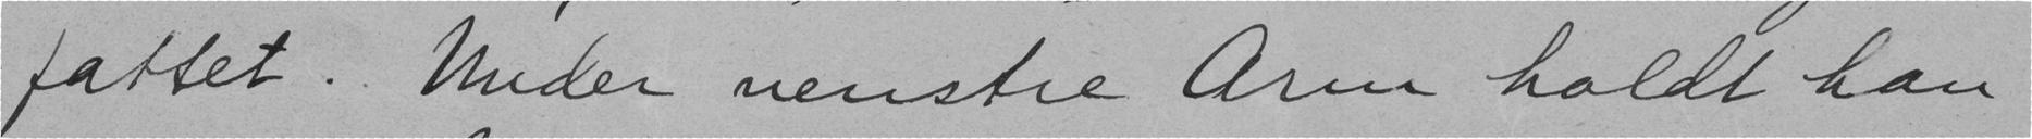fattet. Under venstre Arm holdt han
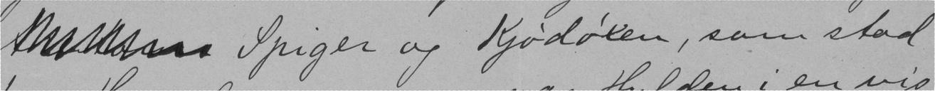Mtaaas Spiger og Kjødøxen, som stod
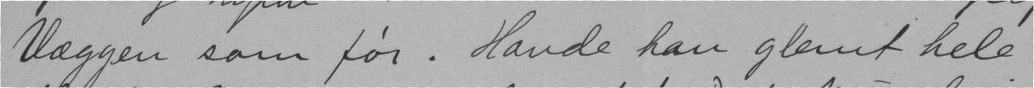Væggen som før. Havde han glemt hele
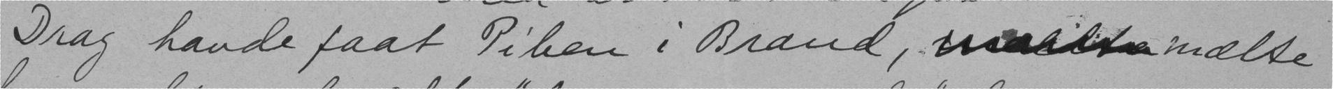Drag havde faat Piben i Brand, mallt mælte
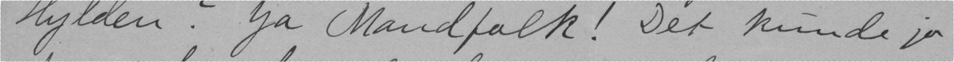Hylden? Ja Mandfolk! Det kunde jo
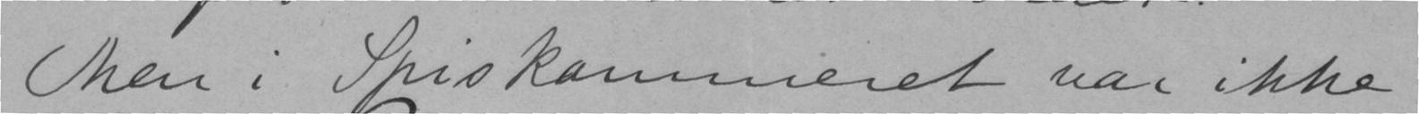Men i Spiskammeret var ikke
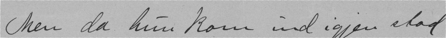Men da hun kom ind igjen stod
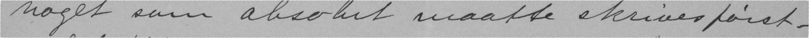noget som absorbet maatte skrives først-
